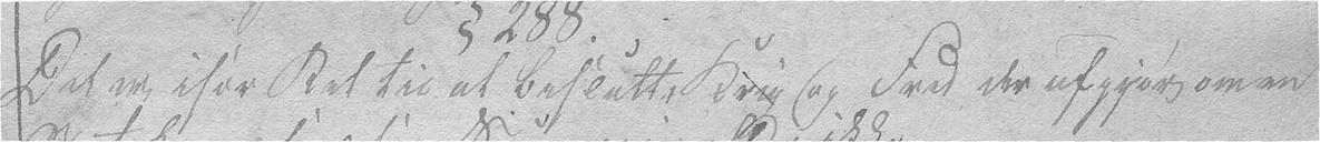Det er især Ret til at beslutte Krig og Fred der afgjør om en
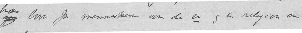jeg love for menneskene som de er og en religion om
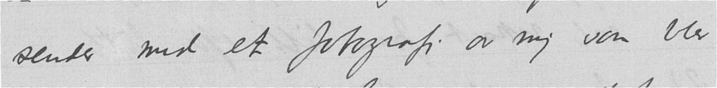Jeg sender med et fotografi av mig som ble
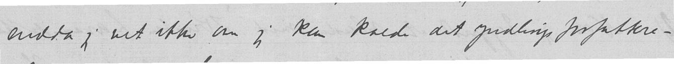- endda jeg vet ikke om jeg kan kalde det yndlingsforfattere - .
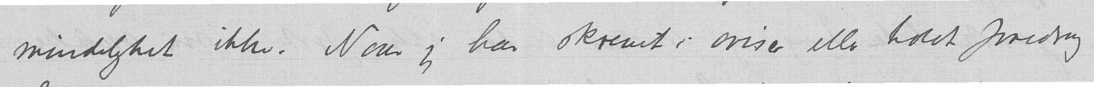mindelighet ikke. Naar jeg har skrevet i aviser eller holdt foredrag
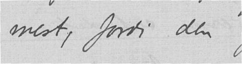mest, fordi den
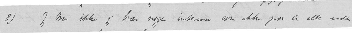8) Jeg tror ikke jeg har nogen interesser som ikke paa en eller anden
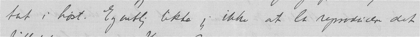tat i høst. Egentlig likte jeg ikke at la reproducere det
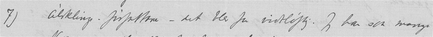7) Älsklings-førfattare - det blir for vidløftig. Jeg har saa mange.
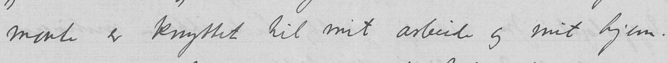maate er knyttet til mit arbeide og mit hjem.
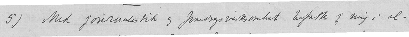5) Med journalistik og foredragsvirksomhet befatter jeg mig i al-
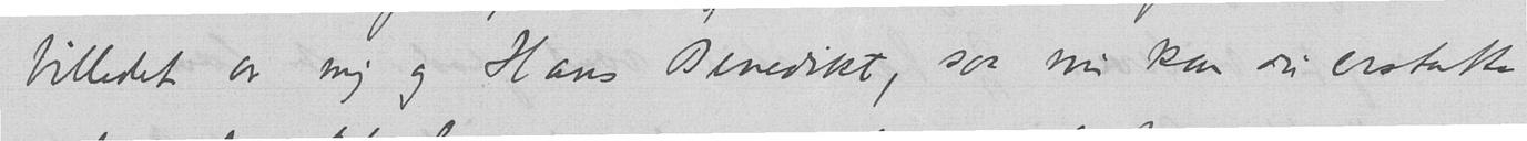billedet av mig og Hans Benedikt, saa nu kan du erstatte
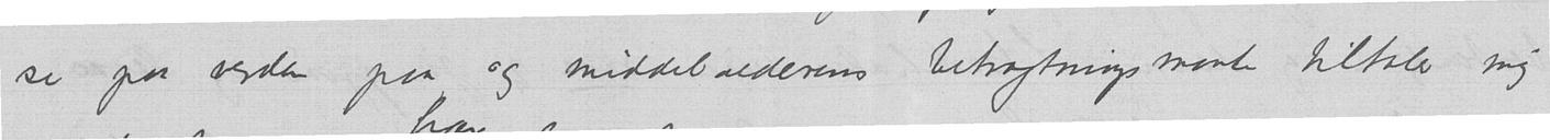se paa verden paa og middelalderens betragtningsmaate tiltaler mig
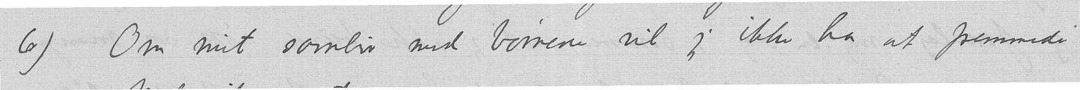6) Om mit samliv med børnene vil jeg ikke ha at fremmede
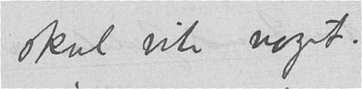skal vite noget.
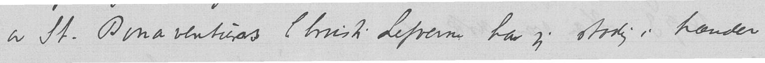av St. Bonaventuras Christi Lefvern har jeg stadig i hænder
